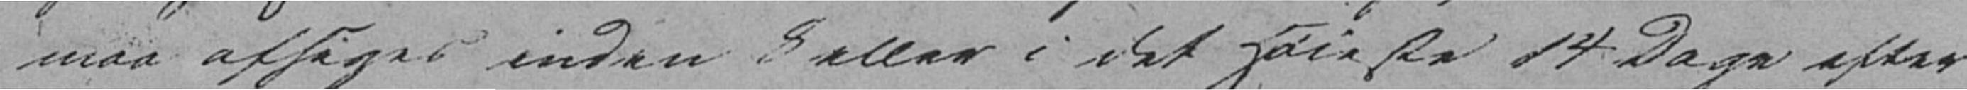maa afsiges inden 8 eller i det høieste 14 Dage efter
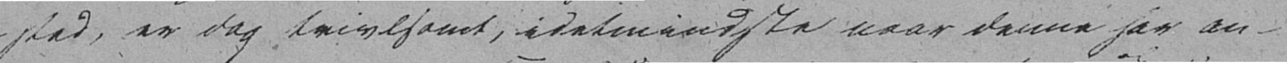sted, er dog tvivlsomt, idetmindste naar denne har an-
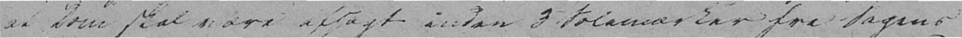at Dom skal være aflagt inden 3 Solemærker fra Sagens
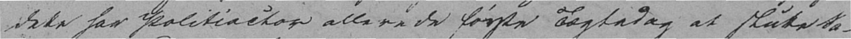dette har Politiactor allerede første Tægtedag at slutte Sa-
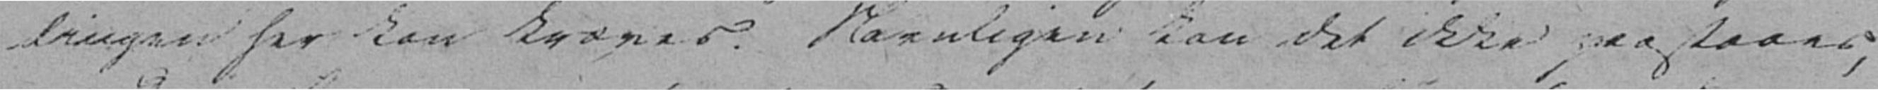lingen her kan kræves. Navnligen kan det ikke paastaaes,
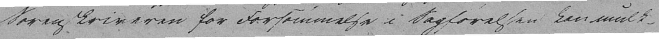Sorenskriveren for Forsømmelse i Sagførelsen kan mulk-
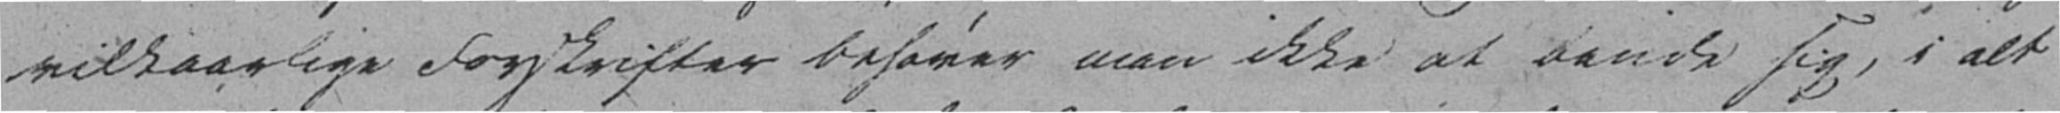vilkaarlige Forskrifter behøver man ikke at binde sig, i alt
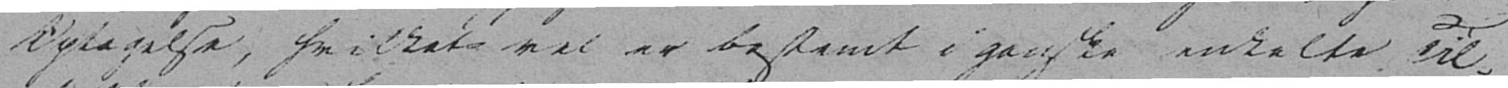Optagelse, hvilket vel er bestemt i ganske enkelte Til-
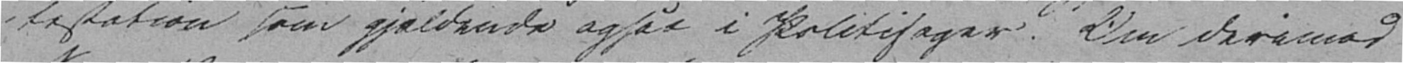testation som gjældende ogsaa i Politisager. Om derimod
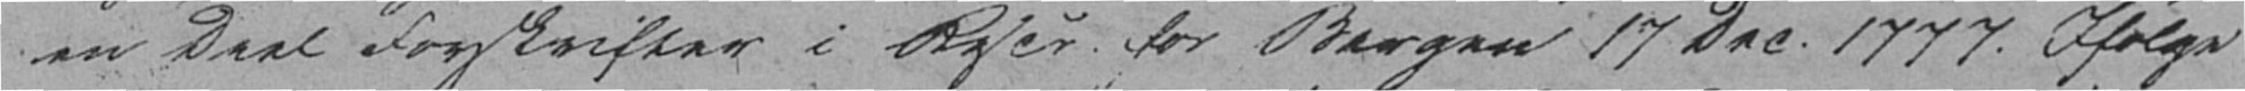en Deel Forskrifter i Rescr. for Bergen 17 Dec. 1777. Ifølge
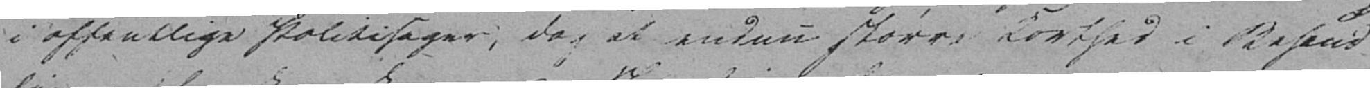i offentlige Politisager, dog at endnu større Korthed i Behand-
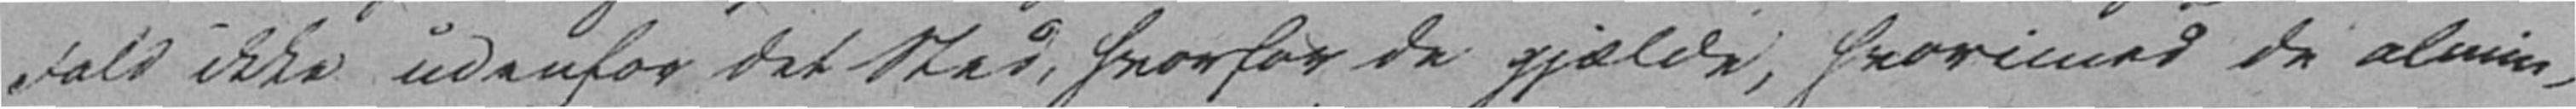Fald ikke udenfor det Sted, hvorfor de gjælde, hvorimod de almin-
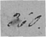360.
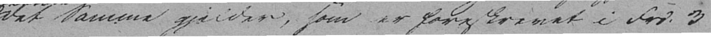det samme gjelder, som er foreskrevet i Frd. 3
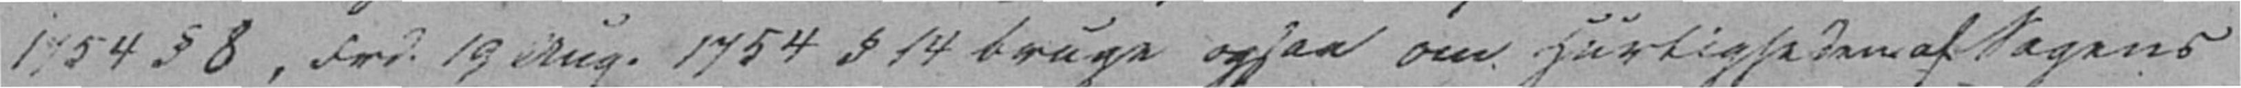1754 § 8, Frd. 19 Aug. 1754 § 14 bruge ogsaa om Hurtigheden af Sagens
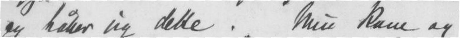og håber jeg dette. Min kone og
